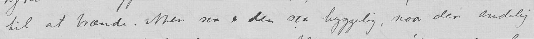til at brænde. Men saa er den saa hyggelig, naar den endelig
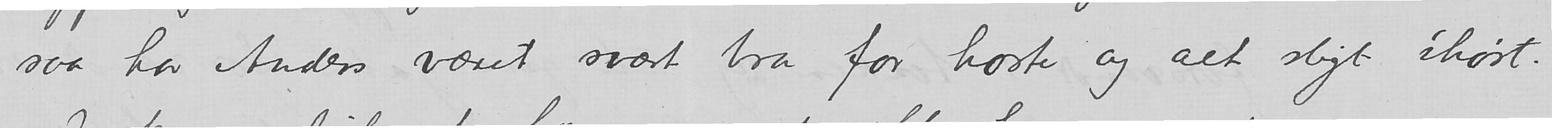– saa har Anders været svært bra for hoste og alt sligt ihøst.
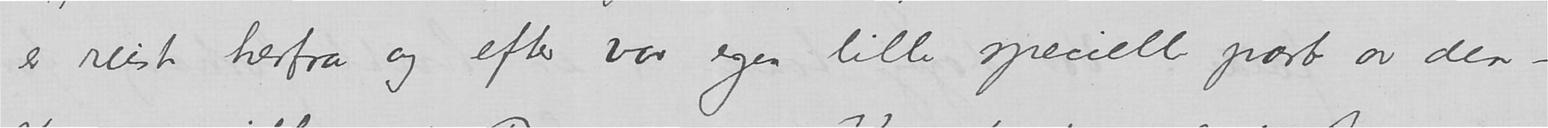er reist herfra og efter vor egen lille specielle part av den –
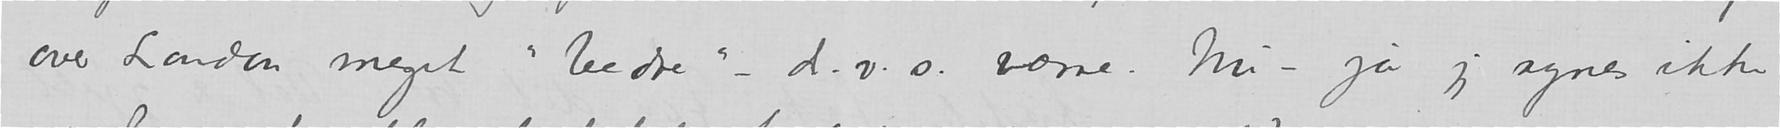over London meget «bedre» – d.v.s. værre. Nu – ja jeg synes ikke
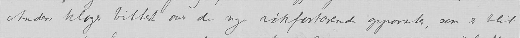Anders klager bittert over de nye røkfortærende apparater, som er blit
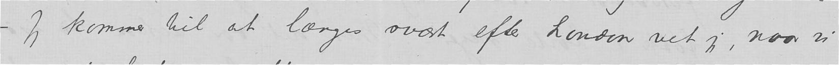– Jeg kommer til at længes svært efter London vet jeg, naar vi
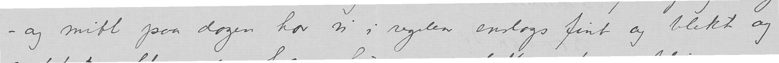– og mit paa dagen har vi i regelen enslags fint og blekt og
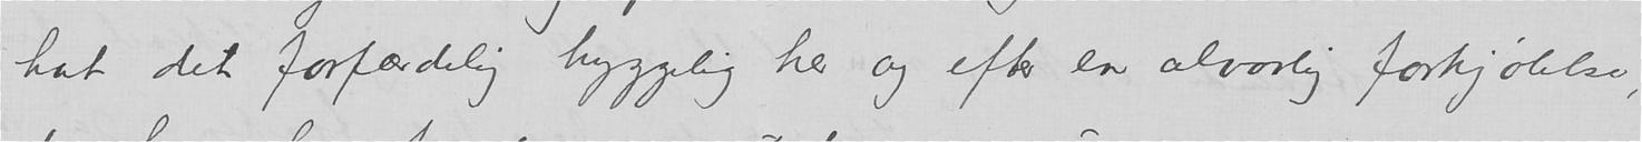hat det forfærdelig hyggelig her og efter en alvorlig forkjølelse,
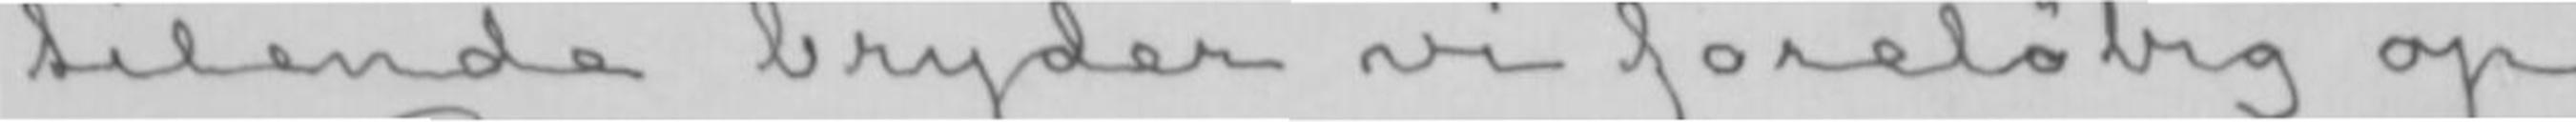tilende bryder vi foreløbig op
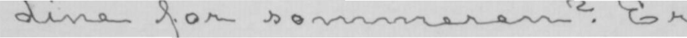og dine for sommeren? Er
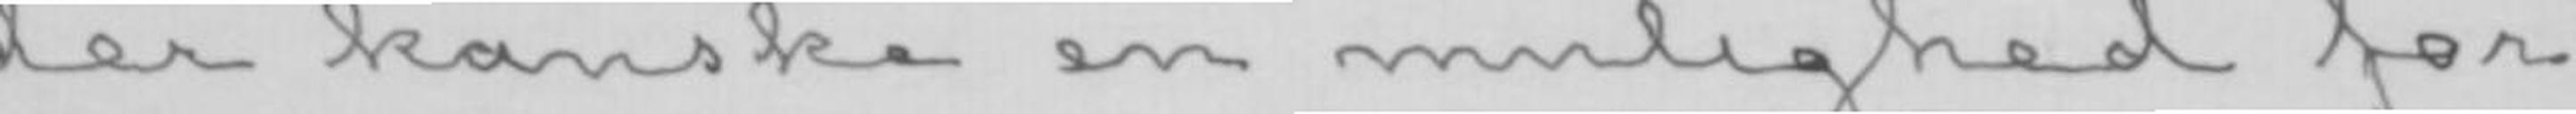der kanske en mulighed for
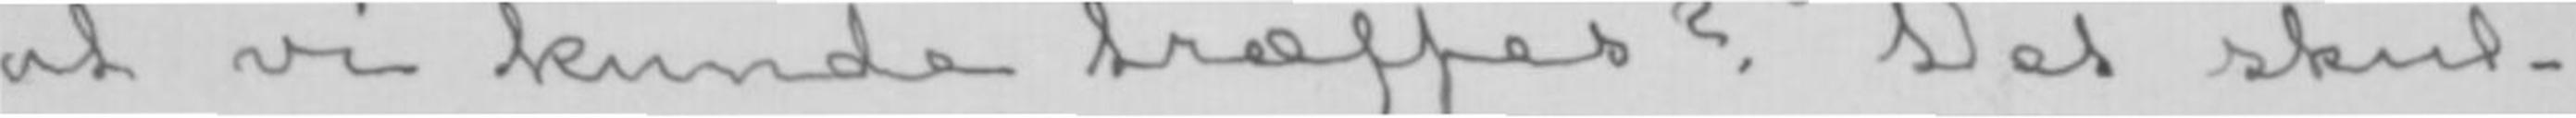at vi kunde træffes? Det skul-
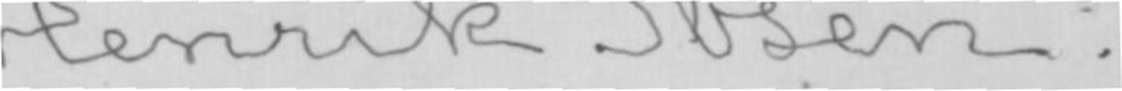Henrik Ibsen.
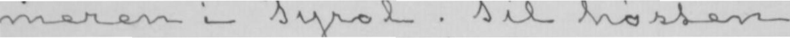meren i Tyrol. Til høsten
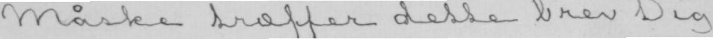Måske træffer dette brev Dig
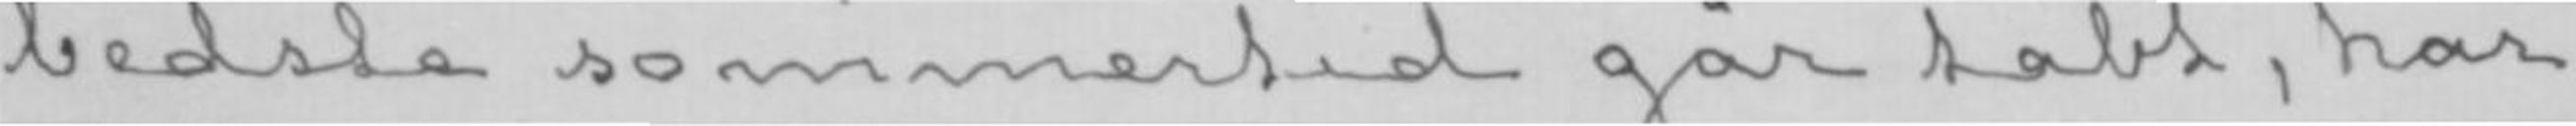bedste sommertid går tabt, har
